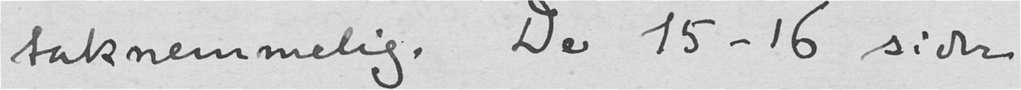taknemmelig. De 15-16 sider
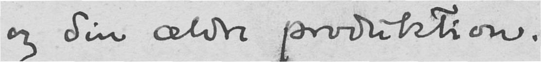og din ældre produktion.
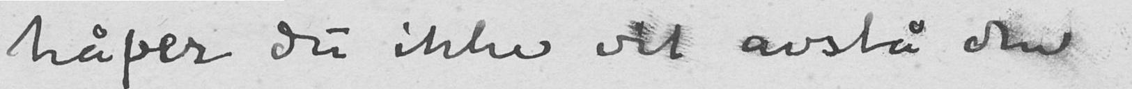håper du ikke vil avslå den
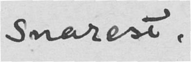snarest.
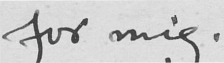for mig.
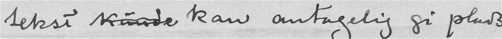tekst kunde kan antagelig gi plads
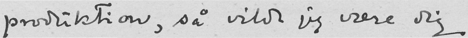produktion, så vilde jeg være dig
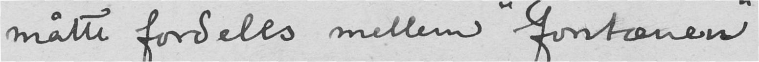måtte fordeles mellem "Fontænen"
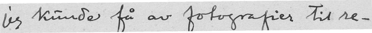jeg kunde få av fotografier til re-
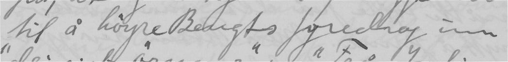til å höyre Bengts fyredrag um
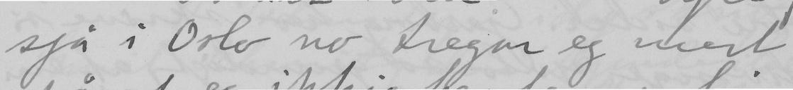sjå i Oslo no tregar eg mest
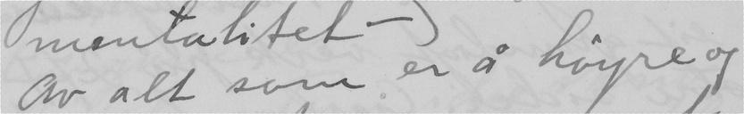Av alt som er å höyre og
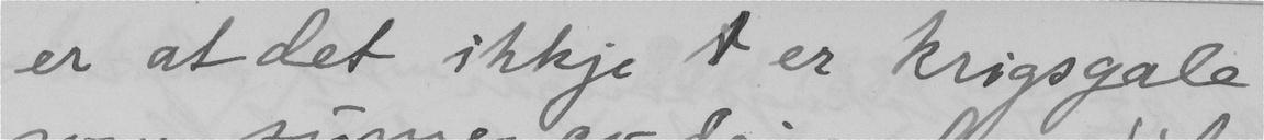er at det ikkje er krigsgale
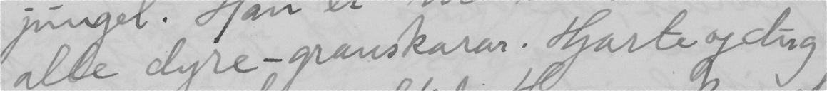alle dyre-granskarar. Hjarte og dug
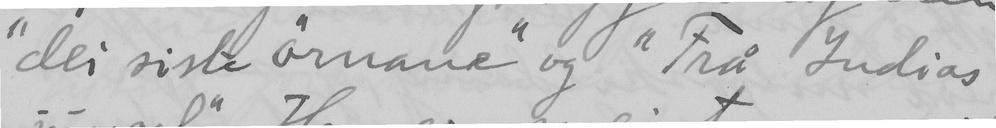"dei siste örnane" og "Frå Indias
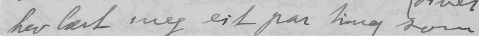hev lært meg eit par ting som
